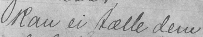kan ei tælle dem
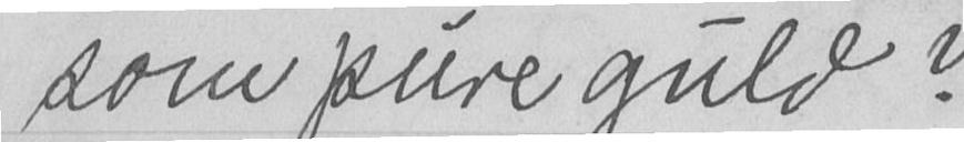som pure guld?
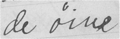de øine
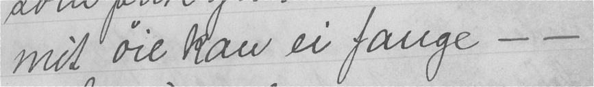mit øie kan ei fange - -
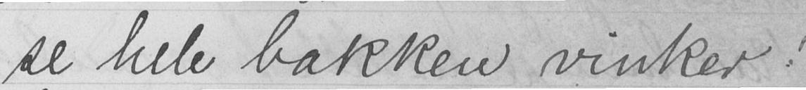se hele bakken vinker!
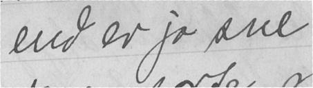end er jo sne
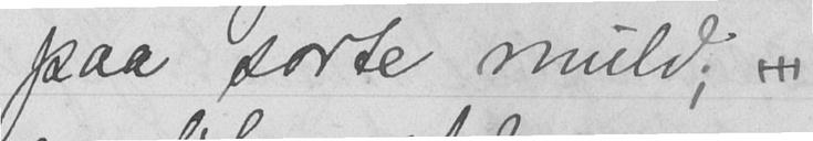paa sorte muld; -
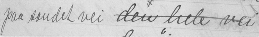paa sandet vei den hele vei
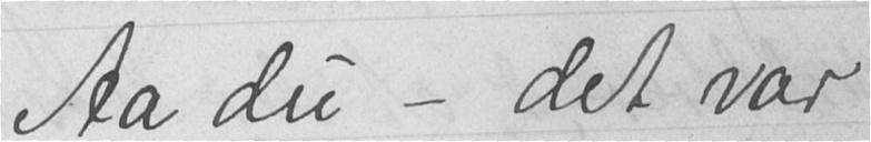Aa du - det var
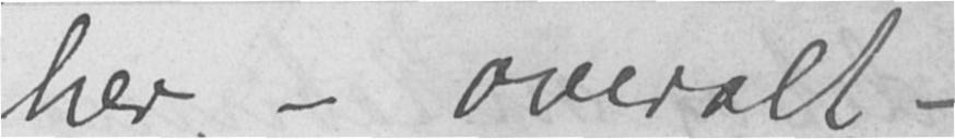her, - overalt -
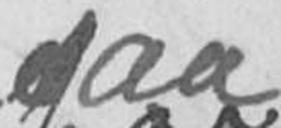saa
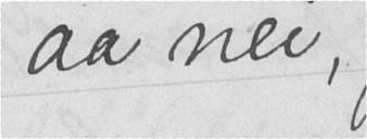aa nei,
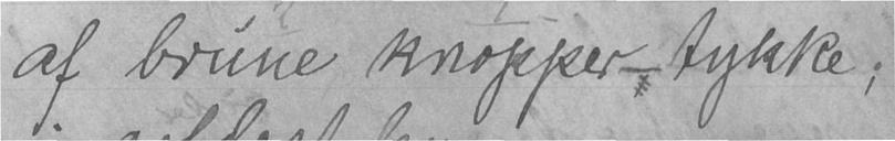af brune knopper - tykke;
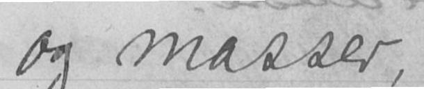og masser,
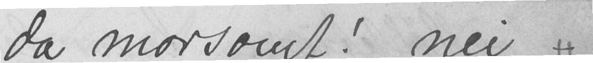da morsomt! nei -
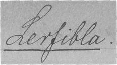Lerfibla.
